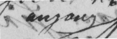engang
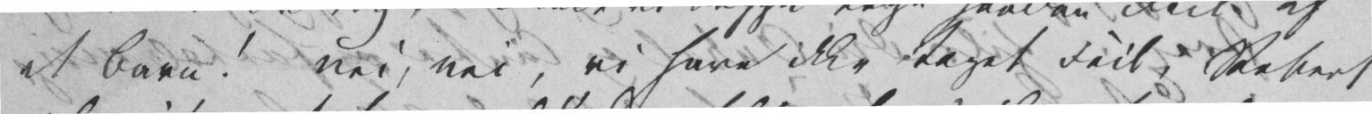et Barn! nei, nei, vi have ikke taget Feil, Robert
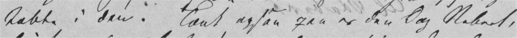tabte i den. Tænk ogsaa paa os den Dag Robert,
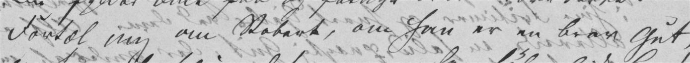Fortæl mig om Robert, om han er en brav Gut,
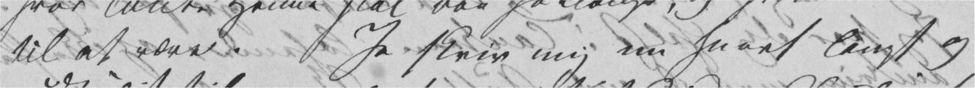til at være. Ja skriv mig nu snart langt og
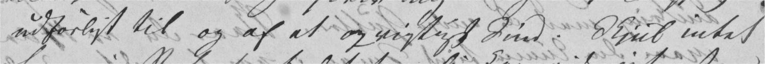udførligt til og af et oprigtigt Sind. Skjul intet
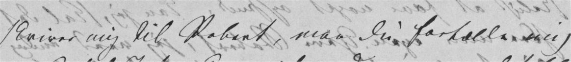skriver mig til Robert, maa Du fortælle mig
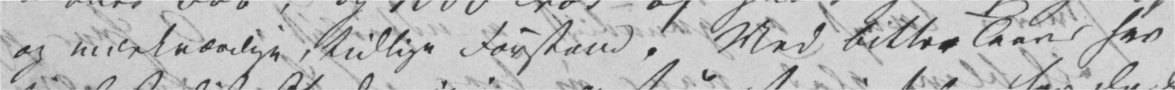og mærkværdige, tidlige Forstand. Med bittre Taarer har
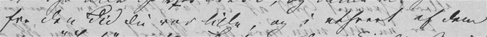fra den Tid Du var lille, og i ethvert af dem
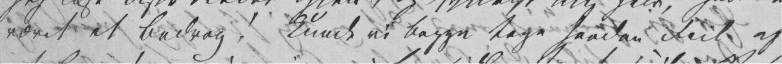været et Bedrag! kunde vi begge tage saadan Feil af
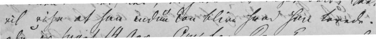vil vise at han endnu kan blive hvad han lovede.
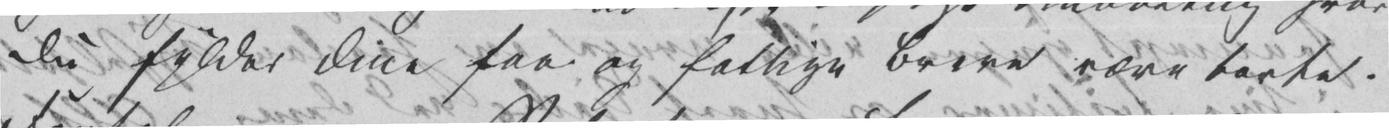Du fylder Dine faa og fattige Breve være borte.
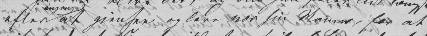efter at gjensee og leve nær sin Mama, for at
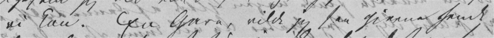vi kan. En Gave, vilde jeg saa gjerne sendt
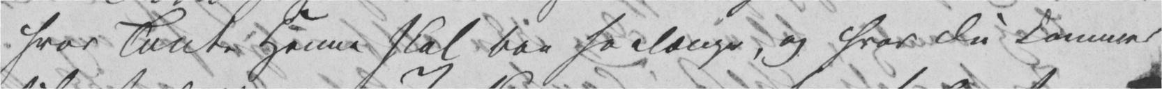hvor Tante Hanne skal boe saalænge, og hvor Du kommer
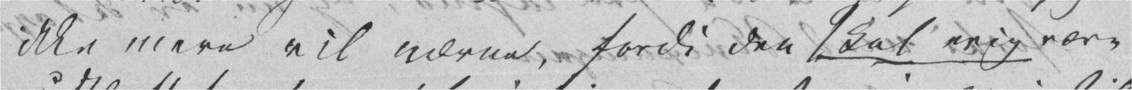ikke mere vil nævne, fordi den skal evig være
